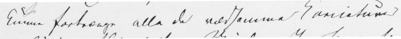kunne fortrænge alle de rædsomme Karicaturer
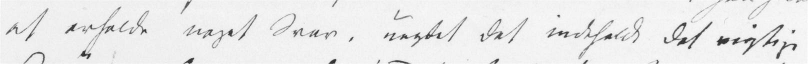at erholde noget Svar, uagtet det indeholdt det vigtige
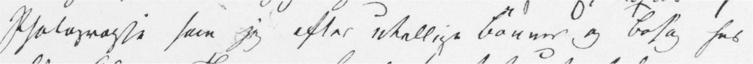Photographie som jeg efter utallige Bønner og Besøg hos
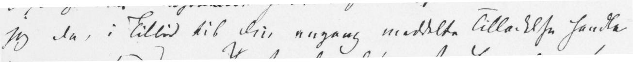jeg da, i Tillid til Din engang meddelte Tilladelse handle
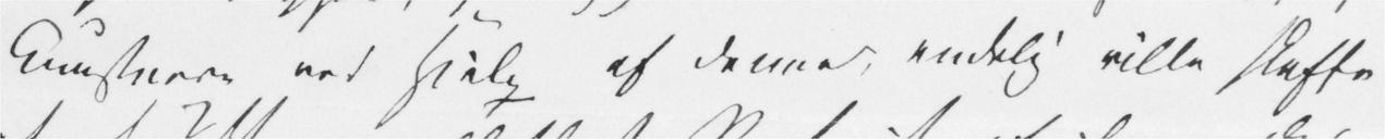Kunstnere ved Hjelp af denne, endelig ville skaffe
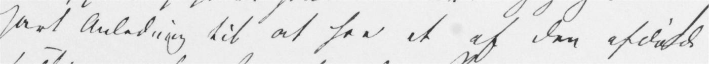havt Anledning til at see et af den afdøde
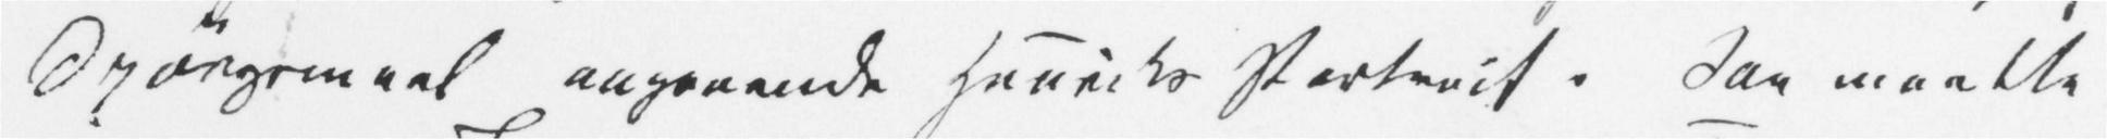Spørgsmaal angaaende Henriks Portrait. Saa maatte
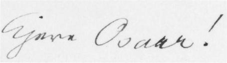Kjere Oscar!
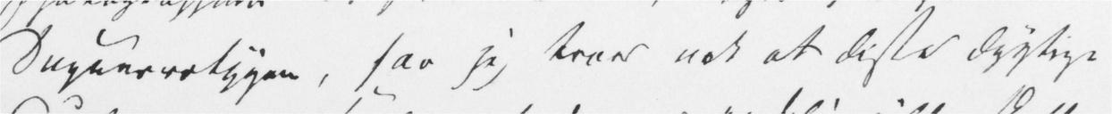Daguerrotypen, saa jeg tror nok at disse dygtige
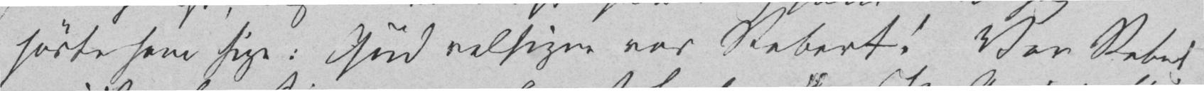hørte ham sige: Gud velsigne vor Robert! Vor Robert
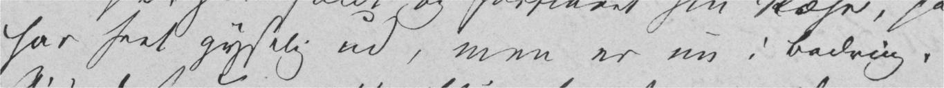har seet gyselig ud, men er nu i Bedring.
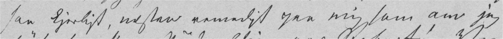saa kjerligt, næsten vemodigt paa mig som om jeg
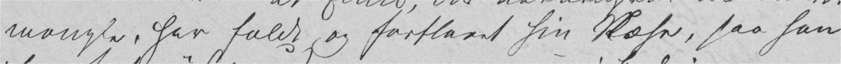mangle, har faldt og forslaaet sin Næse, saa han
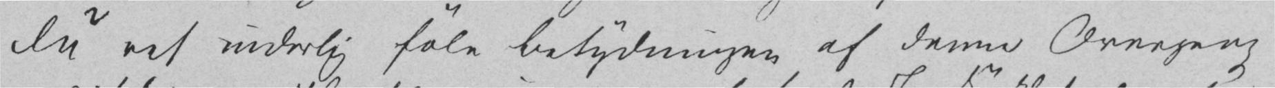Du ret inderlig føle Betydningen af denne Overgang
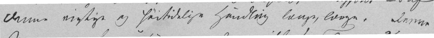denne vigtige og høitidelige Handling længe, længe. Denne
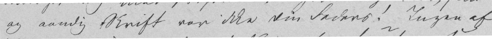og aandig Skrift var ikke Din Faders! Ingen af
